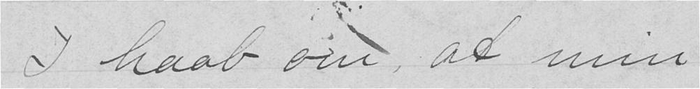I haab om, at min
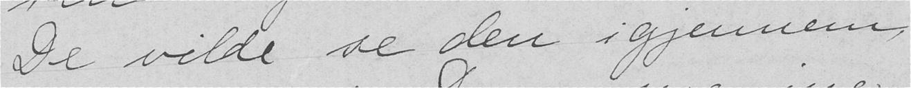De vilde se den igjennem
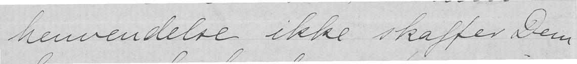henvendelse ikke skaffer Dem
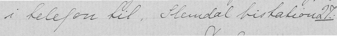i telefon til, Slemdal bistation 27.
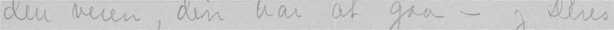den veien, den har at gaa - og Deres
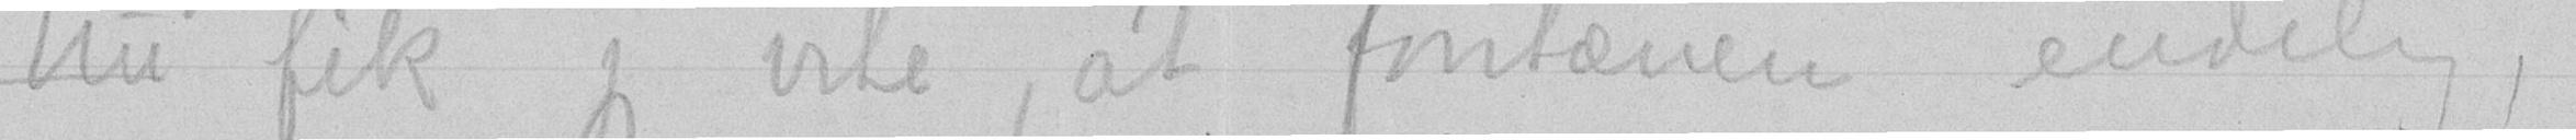Nu fik jeg vite, at fontænen endelig,
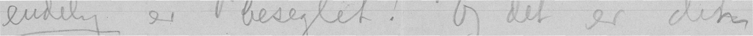endelig er beseglet! Og det er den
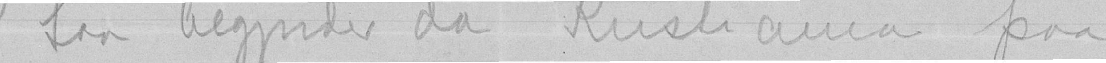Saa begynder da Kristiania paa
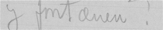og fontænen!
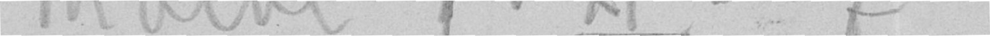Molde 7.XI.07.
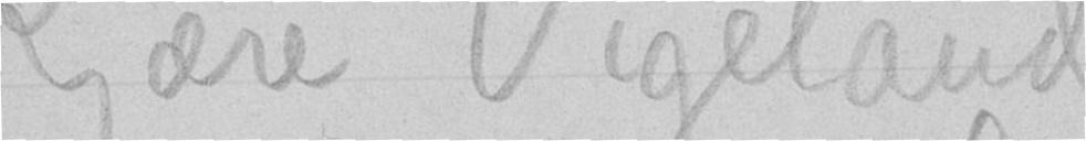Kjære Vigeland
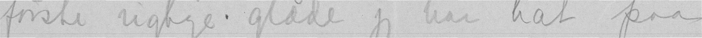første rigtige glæde jeg har hat paa
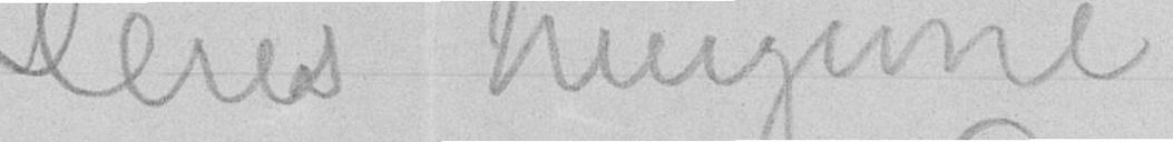Deres hengivne
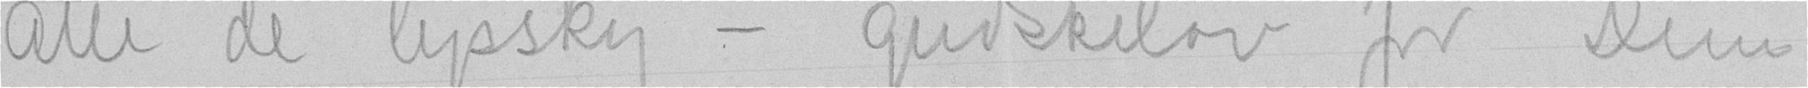alle de lyssky - gudskelob for Dem
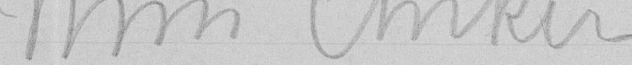Nini Anker
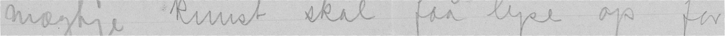mægtige kunst skal faa lyse op for
